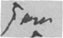som
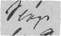Slags
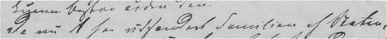Da nu A har udsondret Familien af Staten,
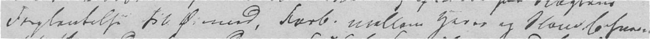Forplantelse til Øiemed, Forb. mellem Herre og Slave Erhverv.
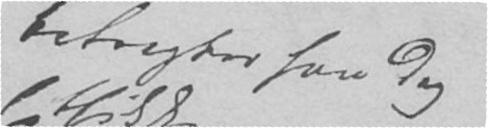betragter han dog
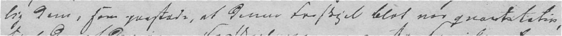lig dem, som paastode, at denne Forskjel blot var qvantitativ,
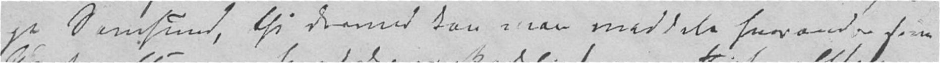ge Samfund, thi dermed han man meddele hverandre sin
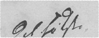det følgte
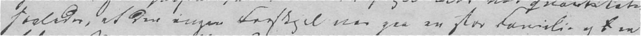saaledes, at der ingen Forskjel var paa en stor Familie og l en
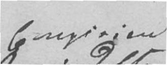Empirien
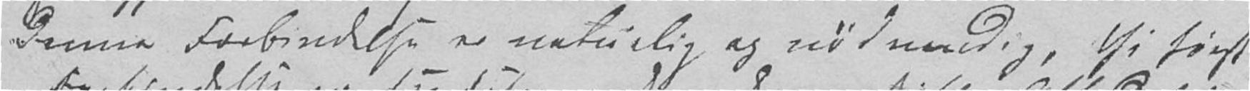Denne Forbindelse er naturlig og nødvendig, thi først
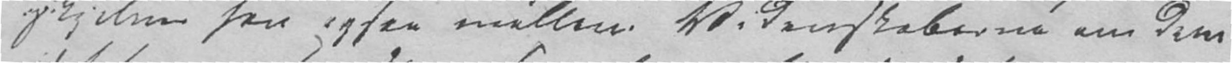skjelner han ogsaa mellem Videnskaberne om dem
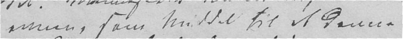evnen, som Middel til at danne
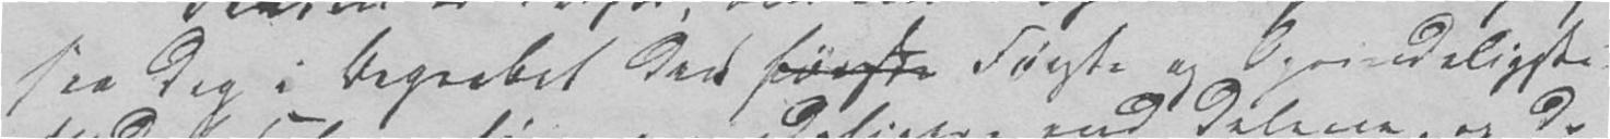saa dog i Begrebet den første Første og Oprindeligste
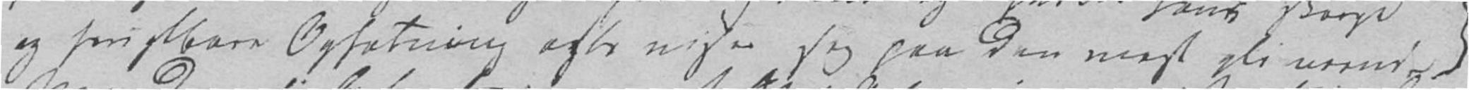og frugtbare Opfatning ofte viser sig paa den mest glimrende
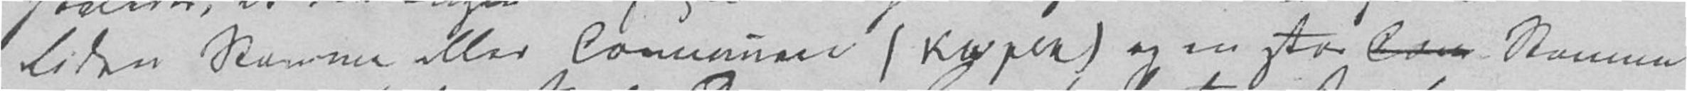liden Stamme eller Commune (typer) og en stor Com Stamme
